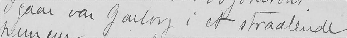Igaar var Garborg i et straalende
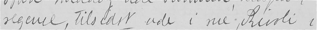segeure, tilsidst ude i rue Rivoli i
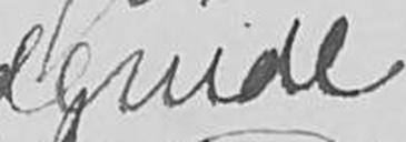derude
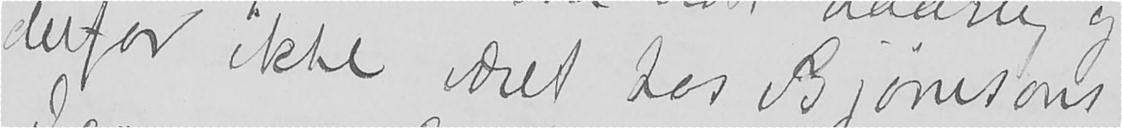derfor ikke været hos Bjørnsons.
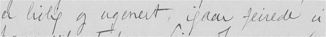er livlig og ugenert, igaar feirede vi
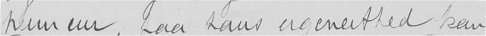humeur, paa hans ugenerthed kan
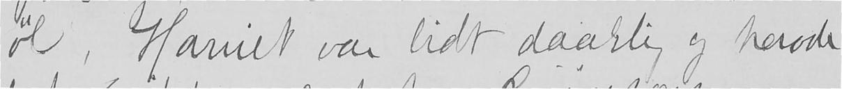øl, Harriet var lidt daarlig og havde
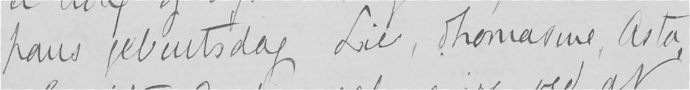hans geburtsdag Lie, Thomasine, Asta,
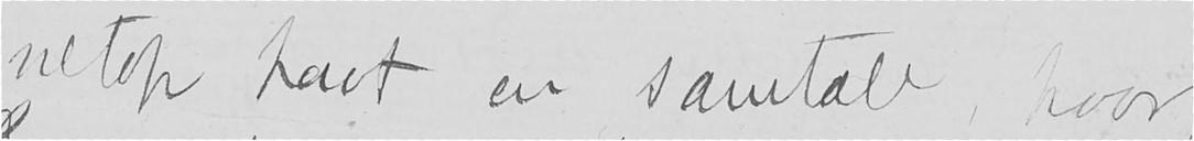netop havt en samtale, hvor
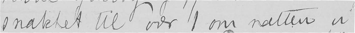snakket til over 1 om natten vi
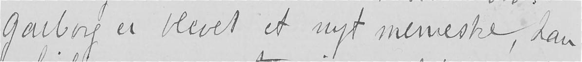Garborg er blevet et nyt menneske, han
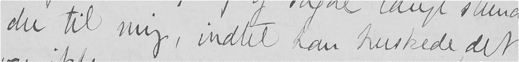du til mig, indtil han huskede, det
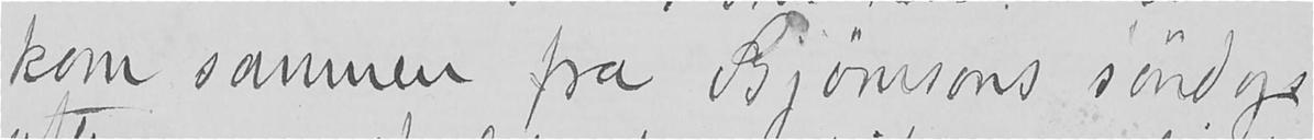kom sammen fra Bjørnsons søndag
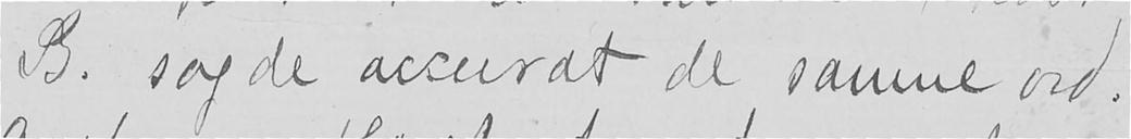B. sagde accurat de samme ord.
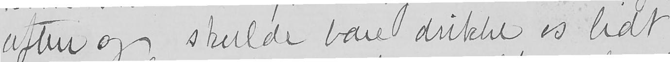aften og skulde bare drikke os lidt
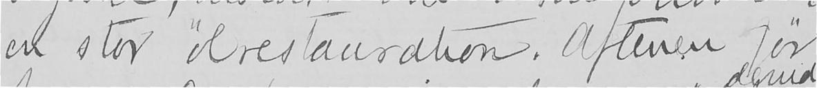en stor ølrestauration. Aftenen før
